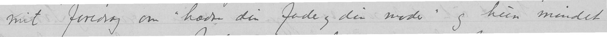mit foredrag om«hædre din fæder og din moder» og hun mindet
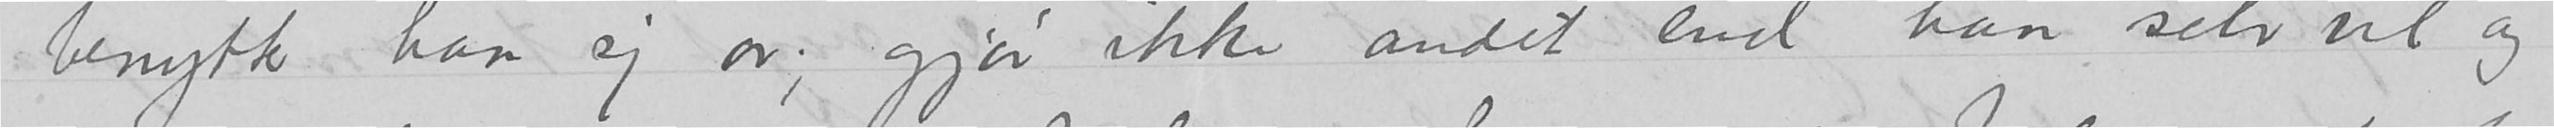benytter han sig av; gjør ikke andet end han selv vil og
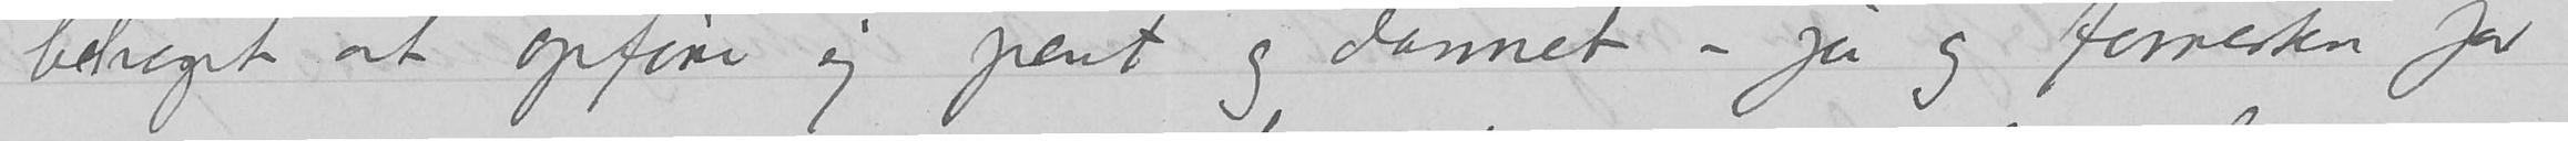behoget at opføre sig pent og dannet – jo og forresten for
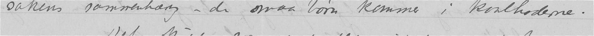sakens sammenhæng ; de smaa børn, kommer i kaalhoderne.
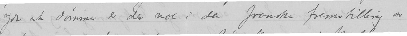gdre at domme er der noe i den franske fremstilling av
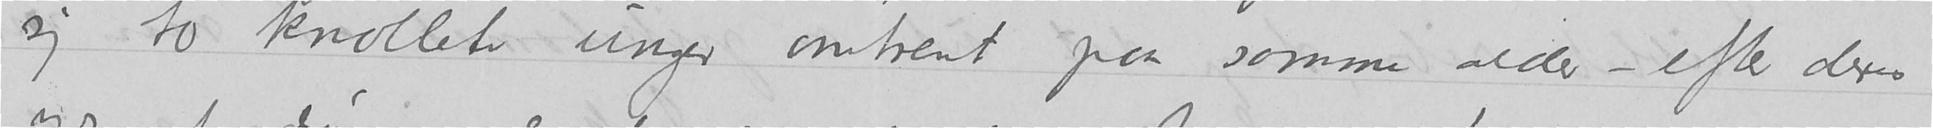sig to knollet unger omtrent paa somme alder – efter deres
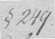§ 249.
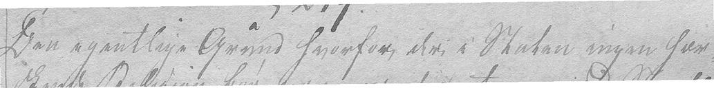Den egentlige Grund, hvorfor der i Staten ingen her-
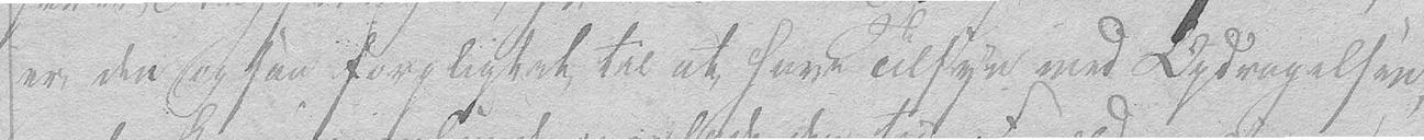er den ogsaa forpligtet til at have Tilsyn med Opdragelsen
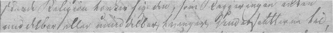middelbar eller umiddelbar tvinger Undersaatterne til,
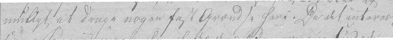muligt at drage nogen fast Grændse heri. Da det interes-
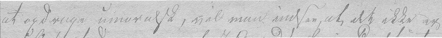at opdrage umoralsk, vil man indsee, at det ikke er
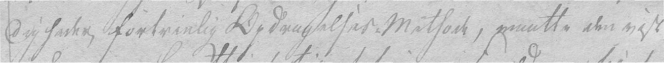digheder fortrinlig Opdragelses-Methode, maatte den vist-
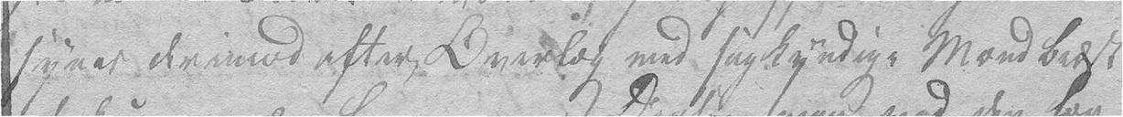synes derimod efter Overlæg med sagkyndige Mænd bedst
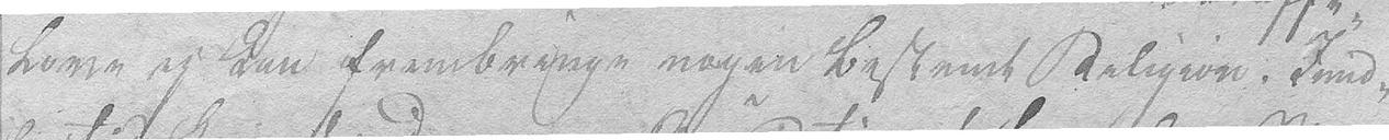love ey kan frembringe nogen bestemt Religion. Imid-
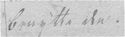benytte den.
